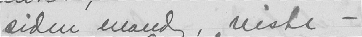siden mandag, reiste -
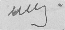mig.
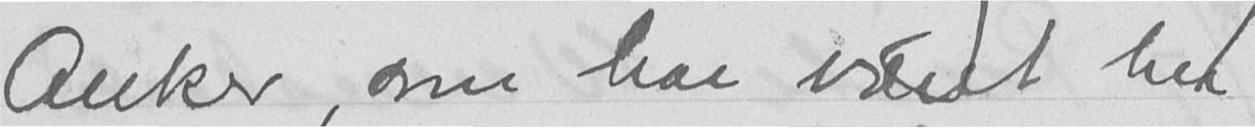Anker, som har været her
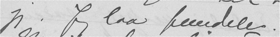jeg. Jeg laa fremdeles.
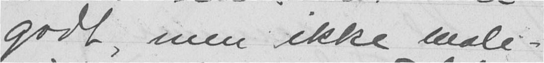godt, men ikke male-
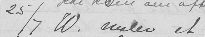25/7 W. maler et
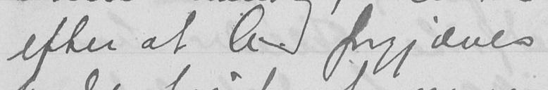efter at C. forgjæves
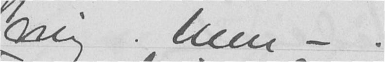mig. Men - .
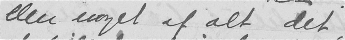eller noget af alt det,
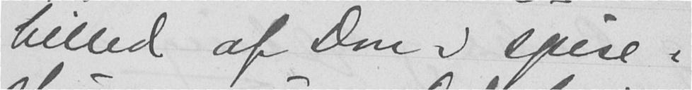billed af Don og spise-
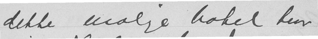dette urolige hotel tror
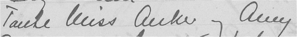Tante Miss Anker og Amy
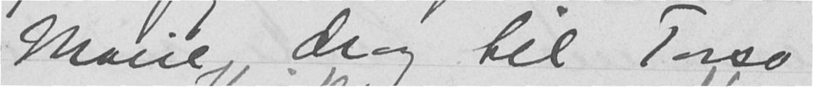Marie drog til Torsø
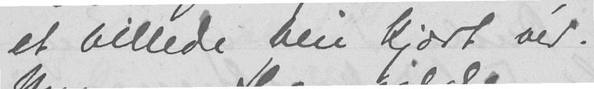et billede blir kjært ved.
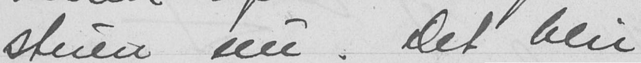stuen nu. Det blir
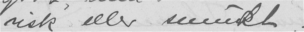risk eller smukt.
